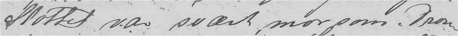Slottet var svært morsom. Dron-
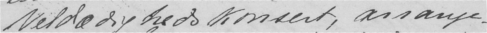Veldædighedskonsert, arrange-
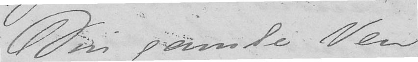Din gamle Ven
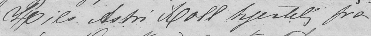Hils Astri Roll hjertelig fra
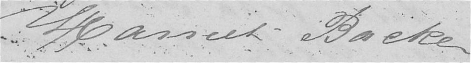Harriet Backer
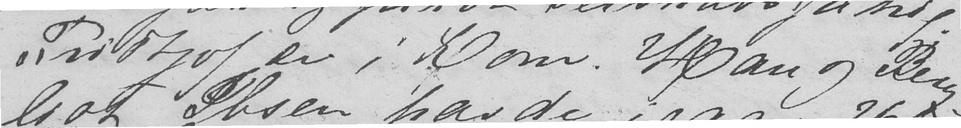Fridtjof er i Rom. Han og Berg-
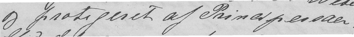og protegeret af Principessaen.
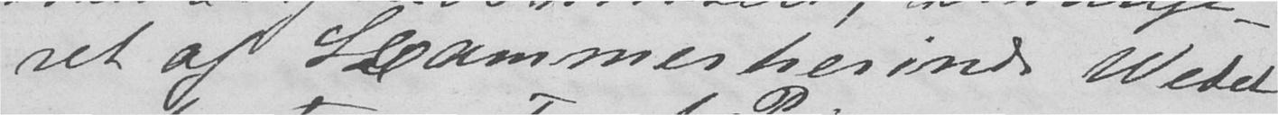ret af Kammerherinde Wedel
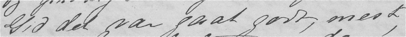Gid det var gaat godt, mest
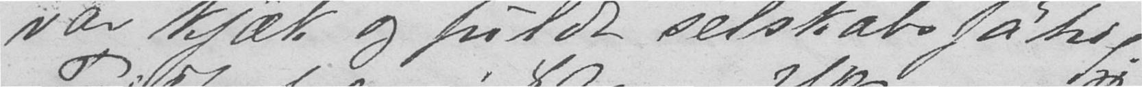var kjæk og fuldt selskabsfahog.
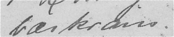bærkrans.
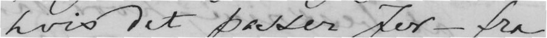hvis det passer Jer - fra
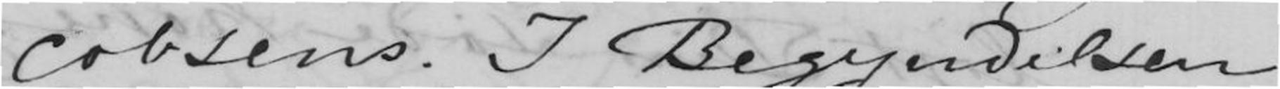cobsens. I Begyndelsen
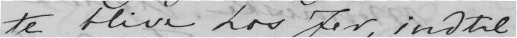te blive hos Jer, indtil
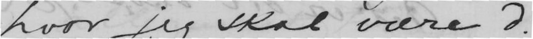hvor jeg skal være d.
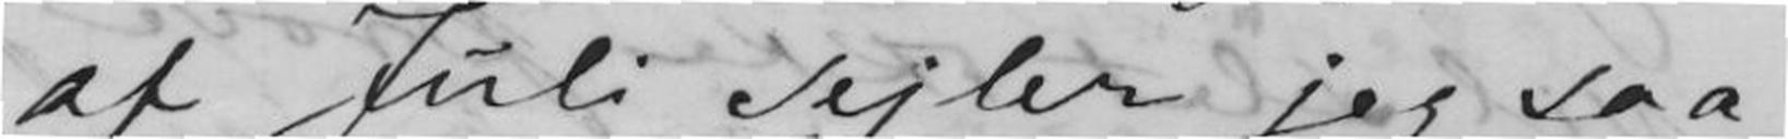af Juli sejler jeg saa -
